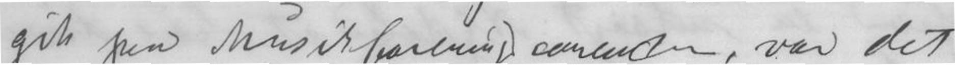gik paa Musikforeningsconcerten, var det
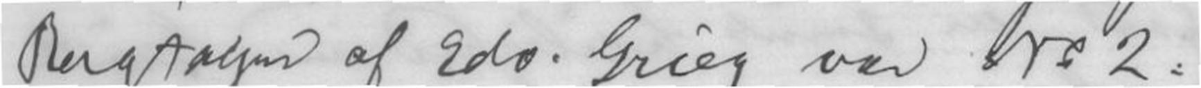Bergtagne af Edv. Grieg var No 2.
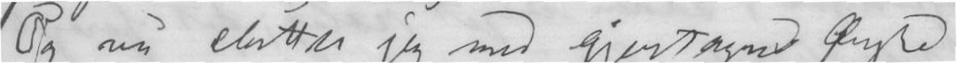Og nu slutter jeg med gjentagne Ønske
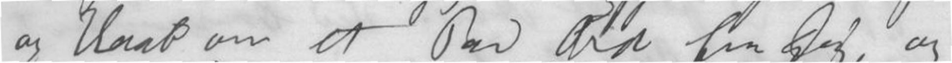og Haab om et Par Ord fra Dig, og
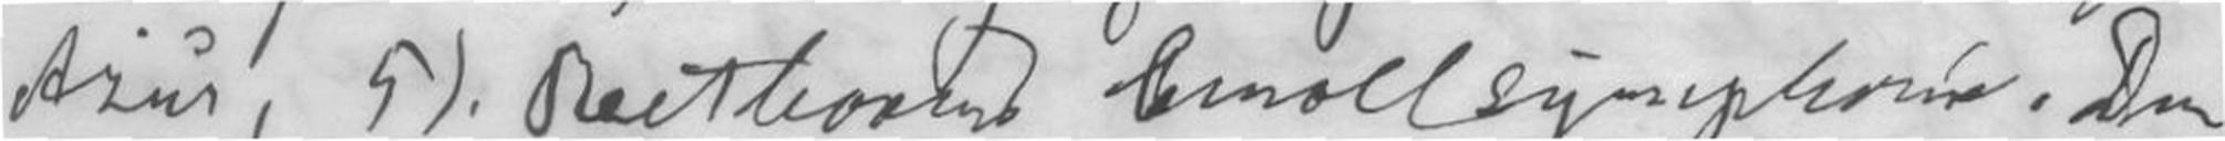Azur, 5) Beethovens Cmollsymphonie. Den
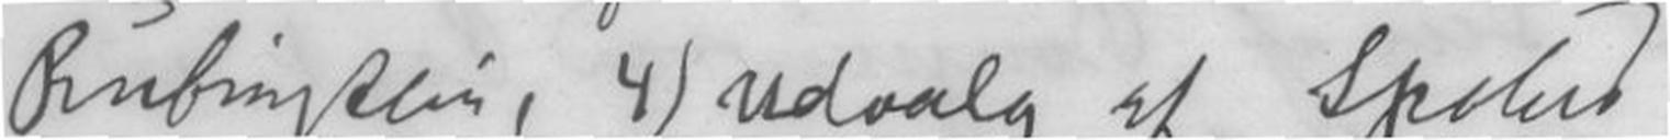Rubinstein, 4) Udvalg af Spolert
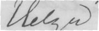Helga
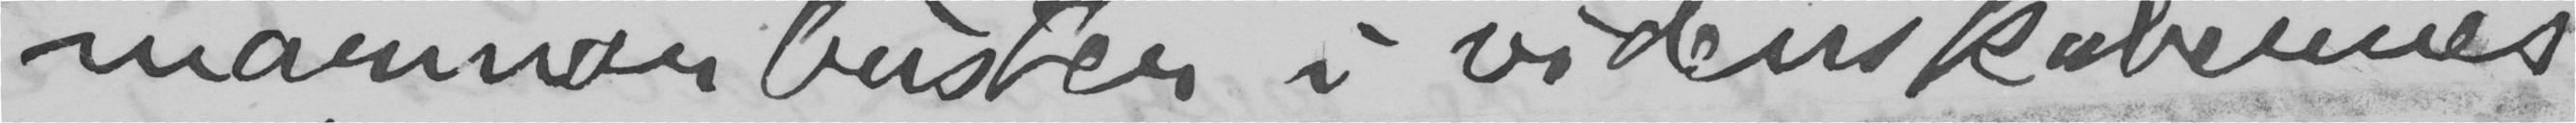marmorbuster i videnskabernes
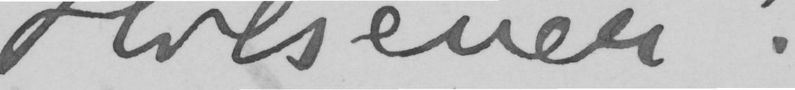Hilsener!
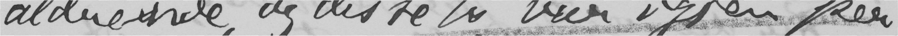aldrende, og disse to var igjen per-
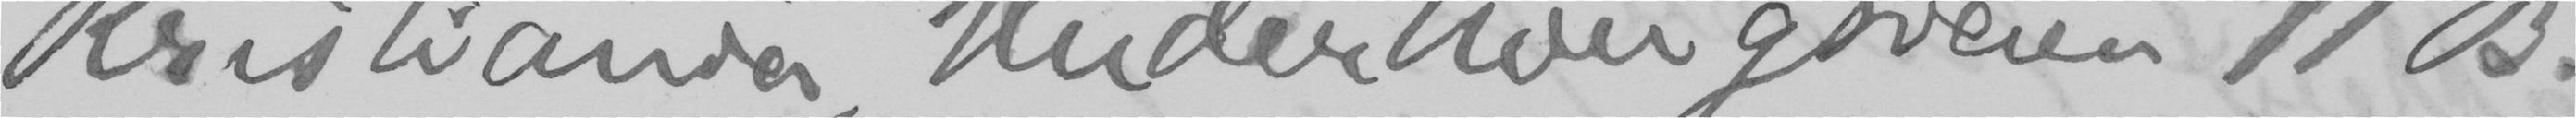Kristiania, Underhaugsveien 11 B.,
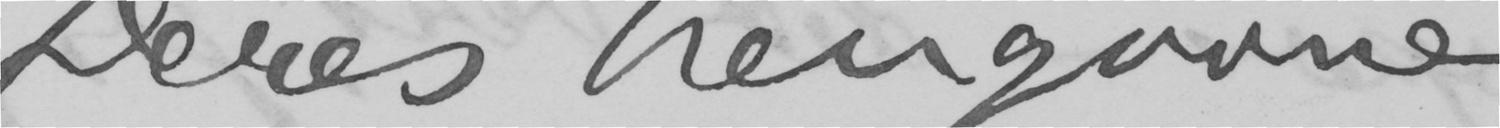Deres hengivne
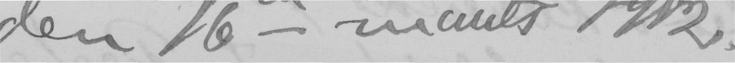den 16de marts 1902.
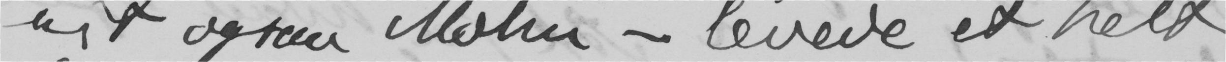rigt ogsaa Mohn – levede et helt
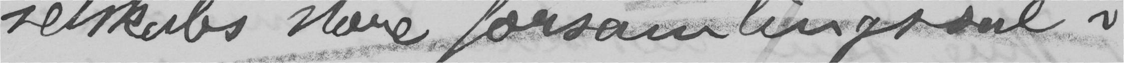selskabs store forsamlingssal i
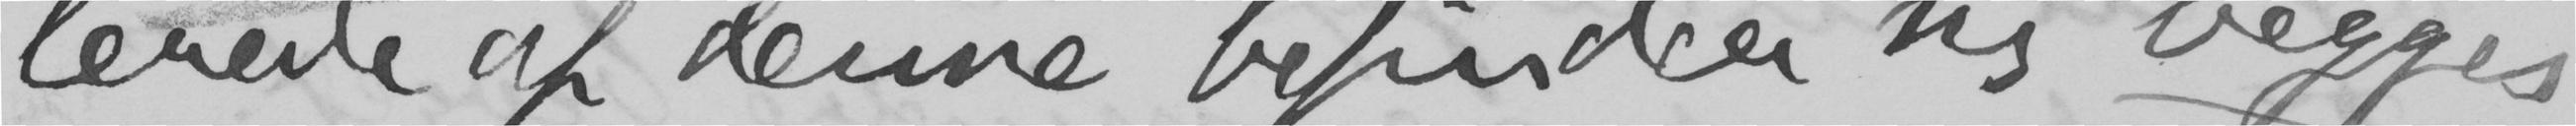lerede af denne befinder sig begges
